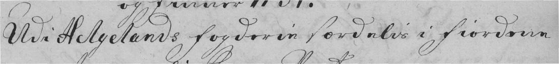Udi Helgelands Fogderie særdelis i Fiordene
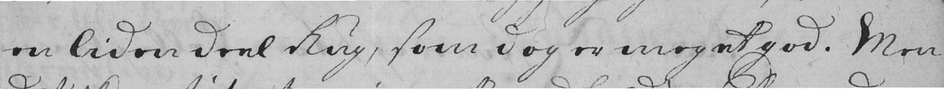en liden deel Rug, som dog er meget god. Men
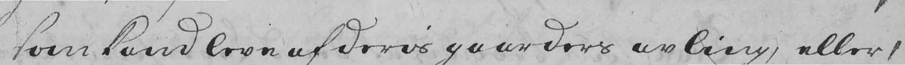som kand leve af deris gaarders avling, eller,
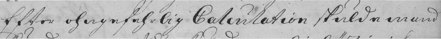Efter ohngefehrlig Calculation skulde mand
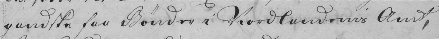gandske faa Bønder i Nordlandenis Amt,
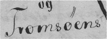Tromsøens
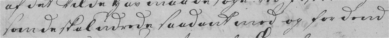som de skal udrede saadant med og for dend
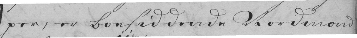per, er boesiddende Nordmænd
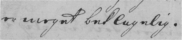er meget beklagelig.
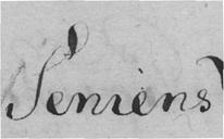Seniens
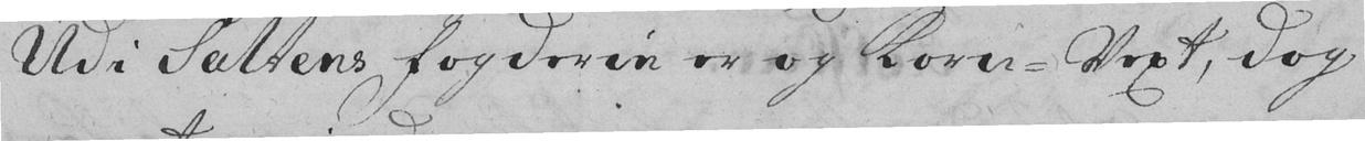Udi Saltens Fogderie er og Korn-Vext, dog
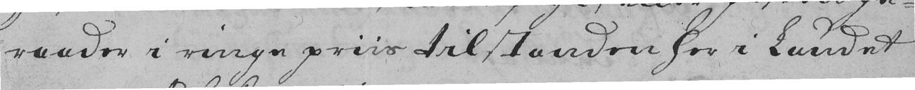raader i ringe priis tilstanden her i Landet
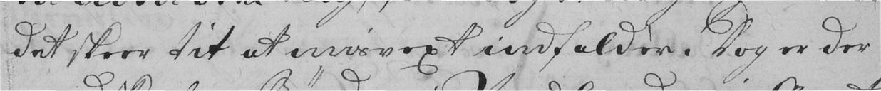det skeer tit at misvext indfalder. Dog er der
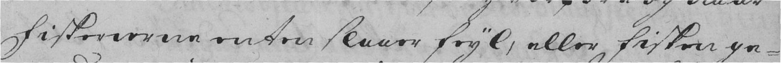fiskerierne enten slaaer feyl, eller fisken ge-
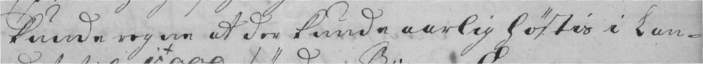kunde regne at der kunde aarlig høstes i Lan-
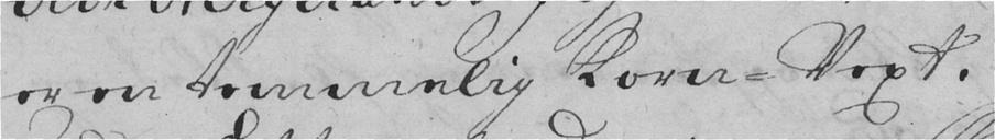er en temmelig Korn-Vext.
